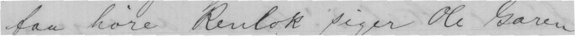faa høre Renlok siger Ole Garen
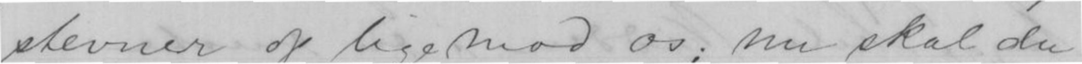stevner op lige mod os; nu skal du
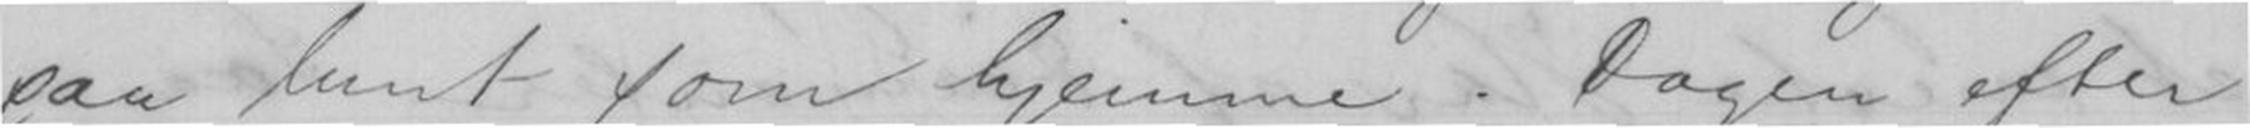saa lunt som hjemme. Dagen efter
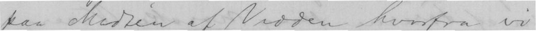paa Midten af Vidden hvorfra vi
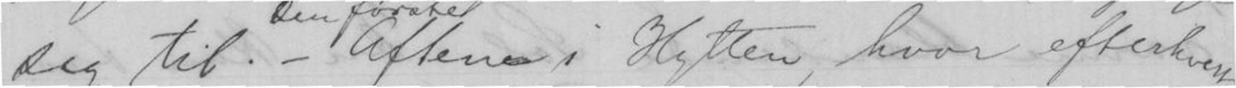sig til. - Aften i Hytten, hvor efterhvert
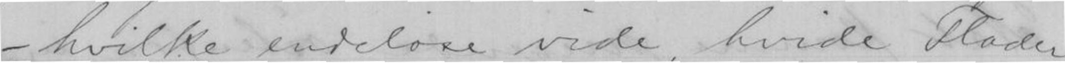- hvilke endeløse vide, hvide Flader
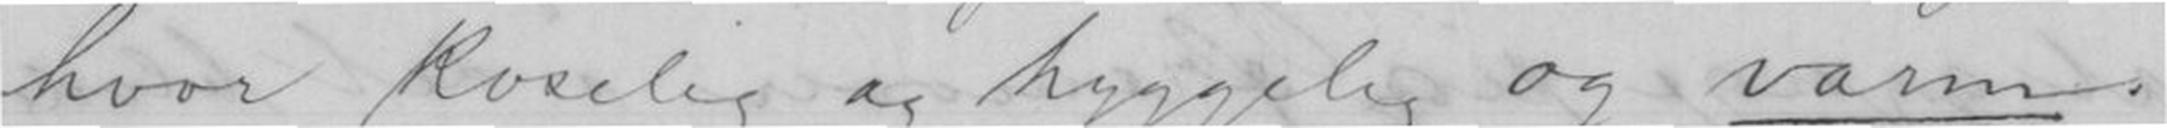hvor koselig og hyggelig og varm.
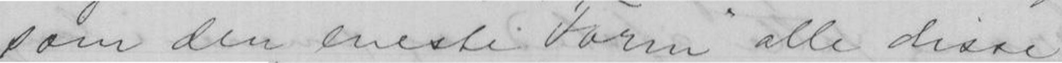som den eneste Form alle disse
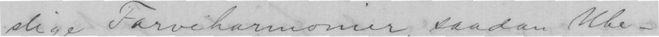slige Farveharmonier, saadan Ube-
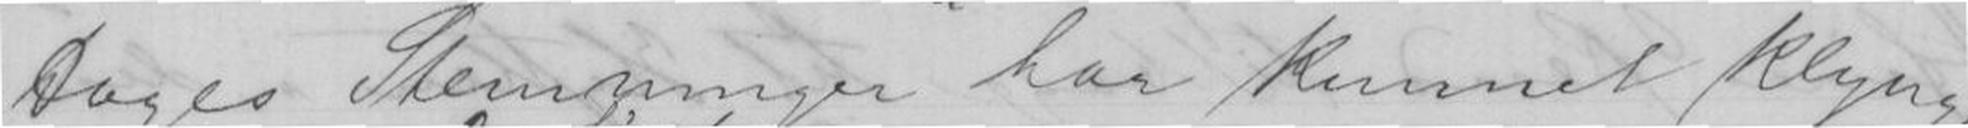Dages Stemninger har kunnet klynge
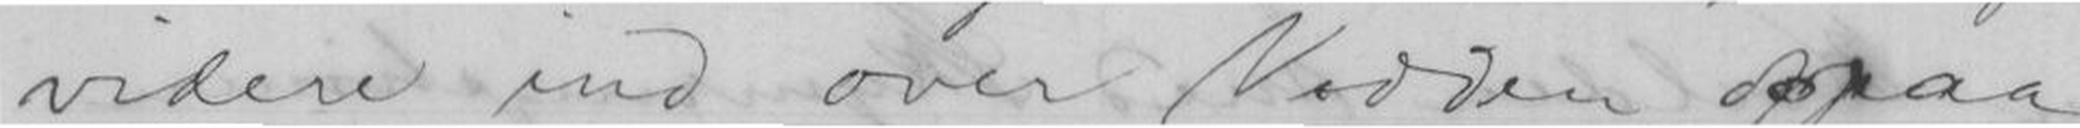videre ind over Vidden oppaa
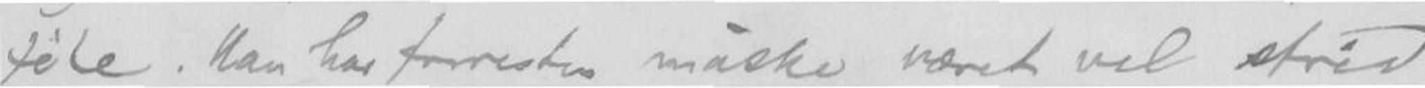føle. Han har forresten måske været vel strid
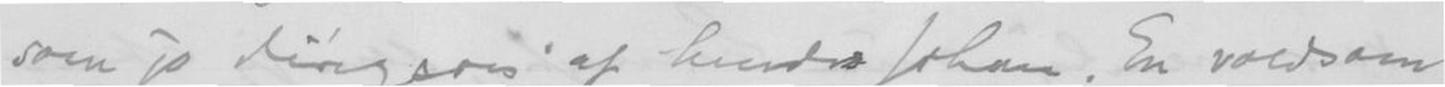som jo dirigeres af hendes Johan. En voldsom
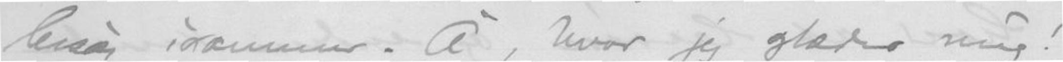besøg isommer. Å, hvor jeg glæder mig!
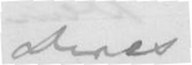Deres
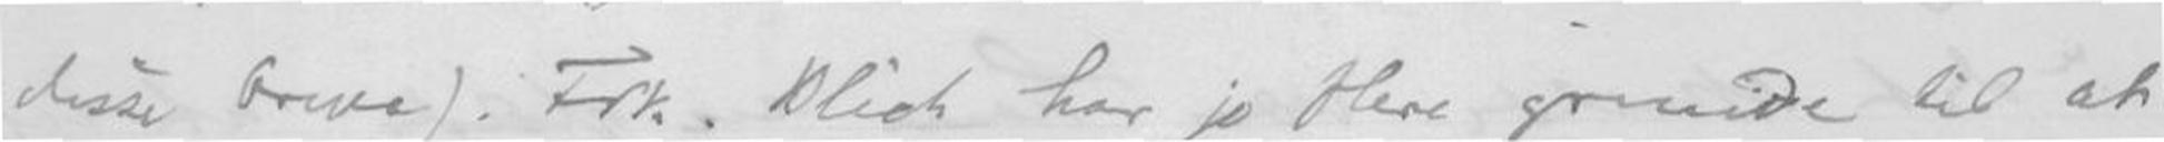disse breve). Frk. Blich har jo flere grunde til at
